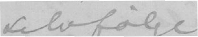selvfølge
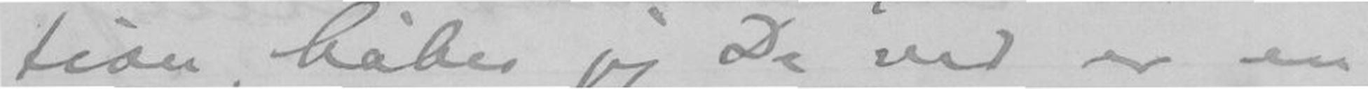tion, håber jeg De ved er en
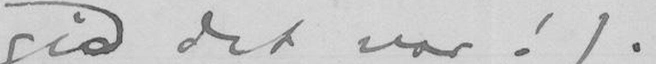gid det var!).
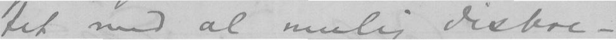tet med al mulig diskre-
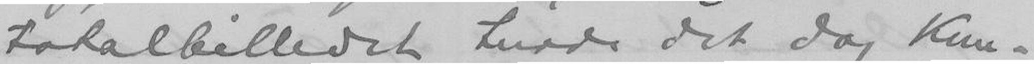totalbildet turde det dog kun-
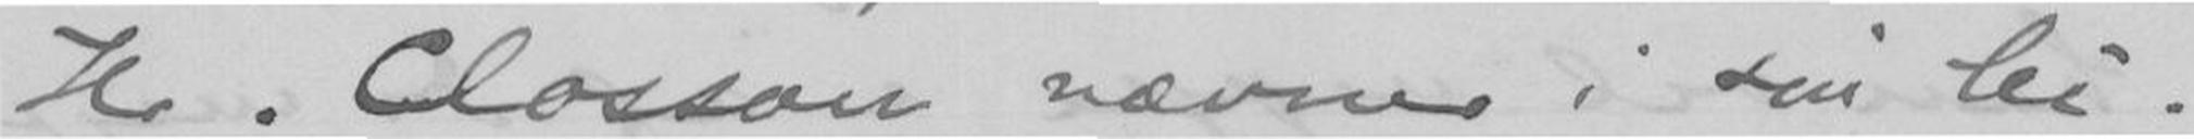Hr. Closson nevnes i sin bi-
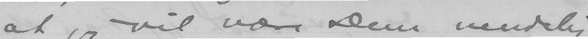at jeg vil være Dem uendelig
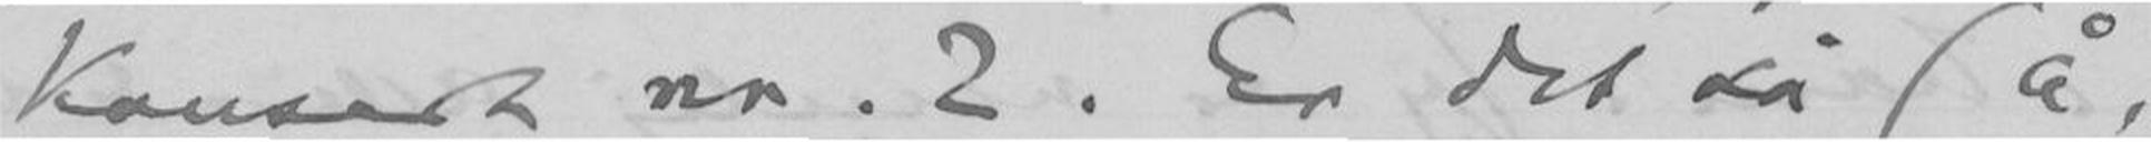Konsert nr. 2. Er det så (å,
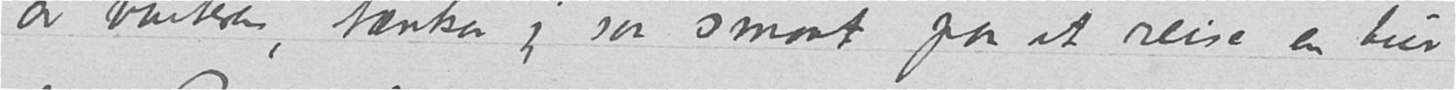av vinteren, tænker jeg saa smaat paa at reise en tur
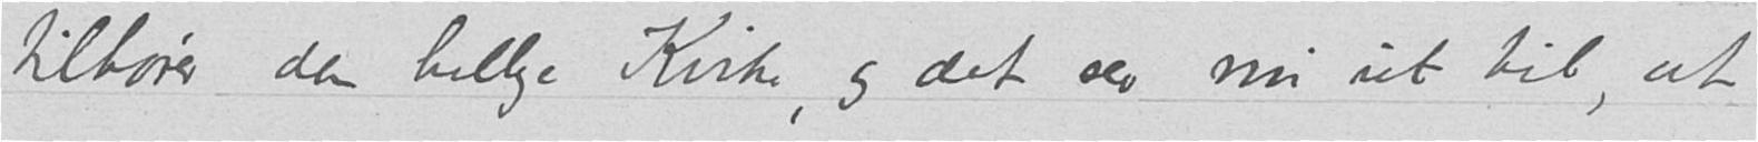tilhører den hellige Kirke, og det ser nu ut til, at
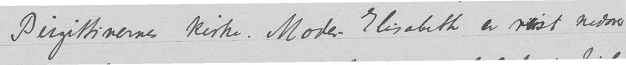Birgittinernes kirke. Moder Elisabeth er reist nedover
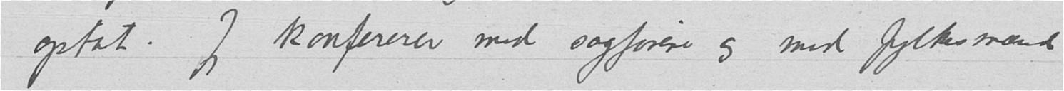optat. Jeg konfererer med sagførere og med fylkesmænd
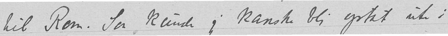til Rom. Saa kunde jeg kanske bli optat ute i
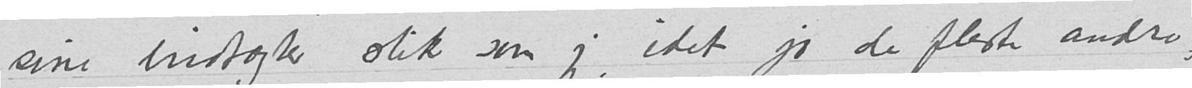sine indtægter slik som jeg, idet jo de fleste andre,
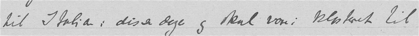til Italien i disse dager og skal være i klosteret til
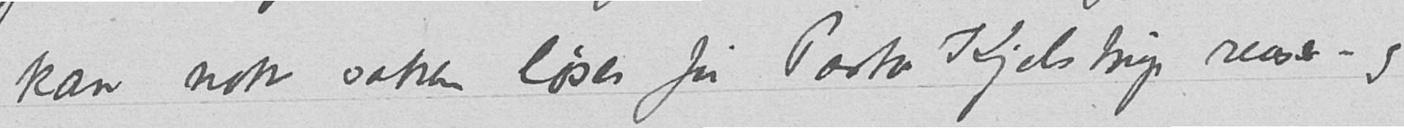kan nok saken løses før Pastor Kjelstrup reiser – og
